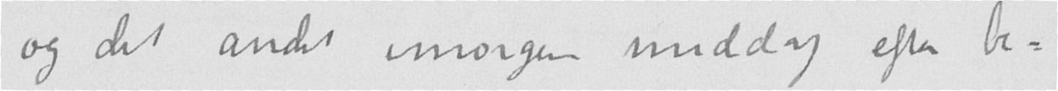og det andet imorgen middag efter be-
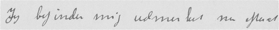Jeg befinder mig udmerket nu efterat
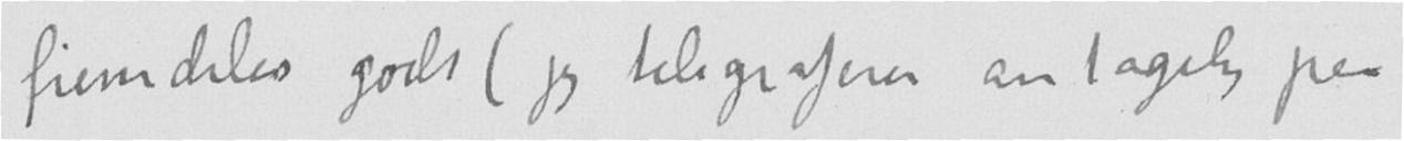fremdeles godt (jeg telegraferer antagelig paa
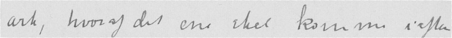ark, hvoraf det ene skal komme i aften
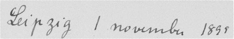Leipzig 1 november 1899
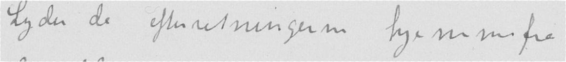Lyder de efterretningerne hjemmefra
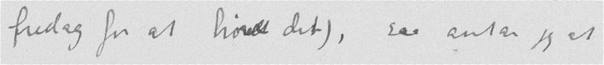fredag for at høre det), saa antar jeg at
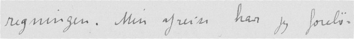regningen. Min afreise har jeg forelø-
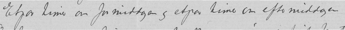Etpar timer om formiddagen og etpar timer om eftermiddagen
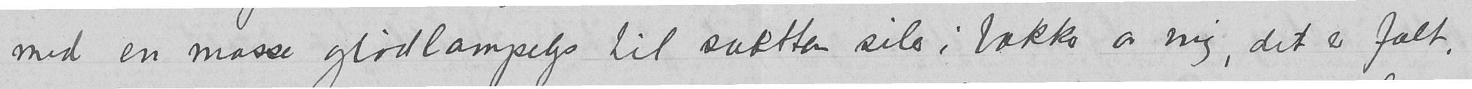med en masse glødlampelys til svætten siler i bakken av mig, det er fælt,
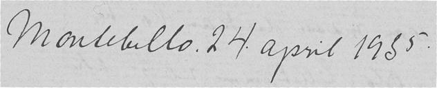Montebello. 24. april 1935.
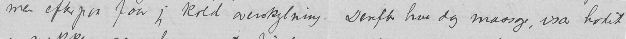men efterpaa faar jeg kold overskylning. Derefter hver dag massage, især hodet
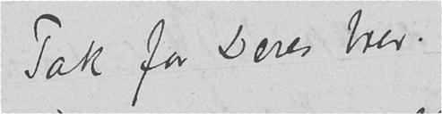Tak for Deres brev.
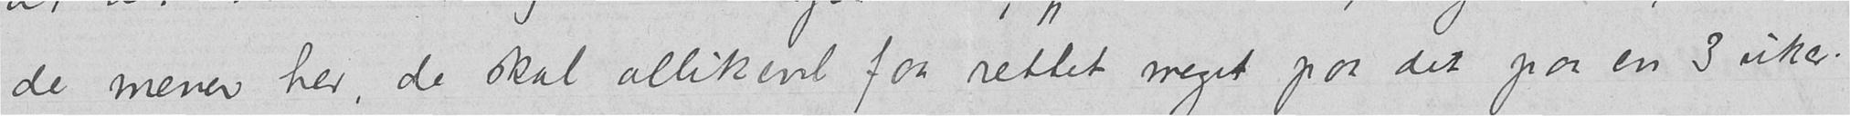de mener her de skal allikevel faa rettet meget paa det paa en 3 uker.
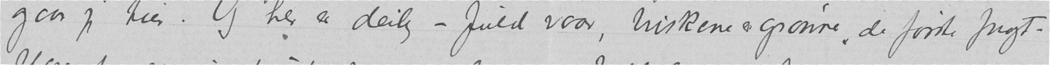gaar jeg tur. Og her er deilig – fuld vaar, buskene er grønne, de første frugt-
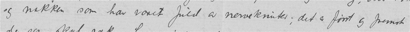og nakken som har været fuld av nerveknuter; det er først og fremst
